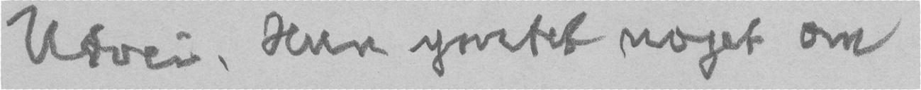Utvei. Hun ymtet noget om
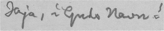Jaja, i Guds Navn!
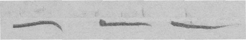- - -
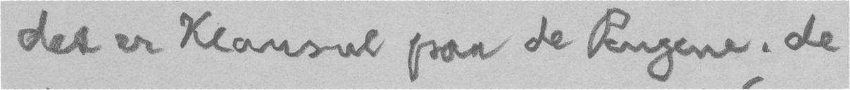det er Klausul paa de Pengene, de
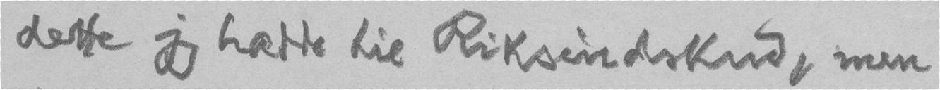dette jeg hadde til Riksindskud, men
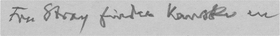Fru Stray finder kanske en
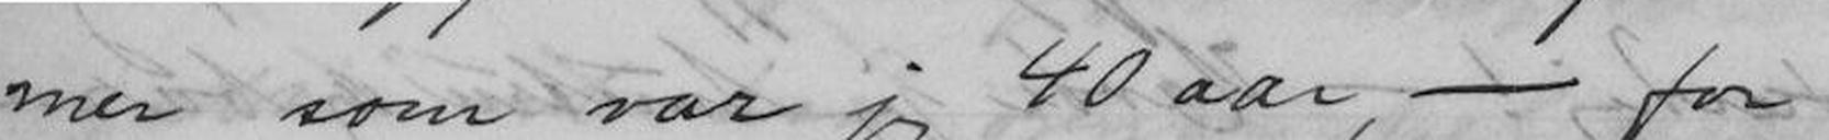mer som var jeg 40 aar, - for
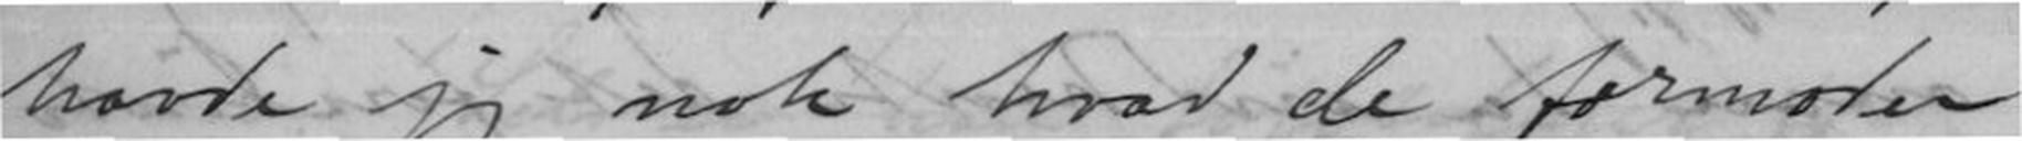havde jeg nok hvad de formoder
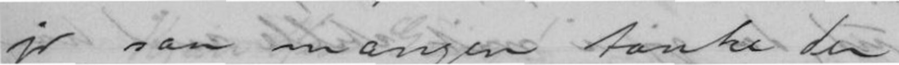jo saa mangen tanke der
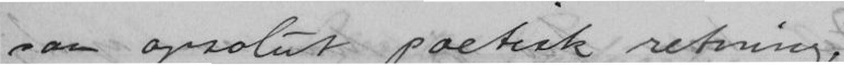saa apsolut poetisk retning,
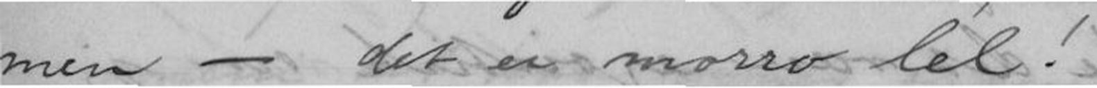men - det er morro lel!
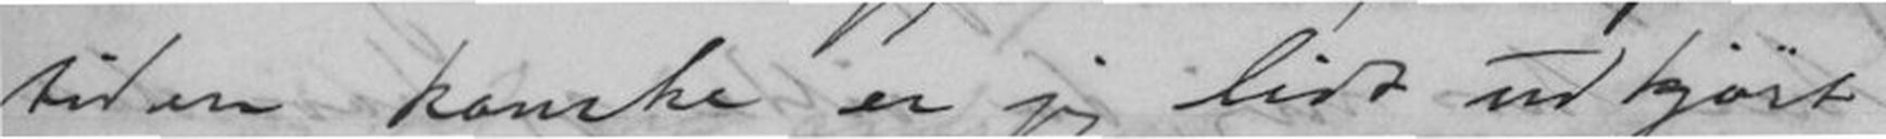tiden kanske er jeg lidt udkjørt
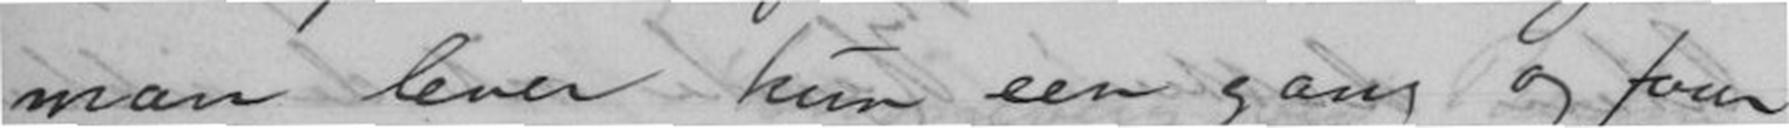man lever kun een gang og faar
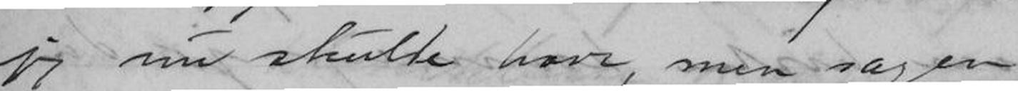jeg nu skulde have, men sagen
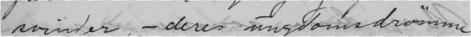svinder, - deres ungdomsdrømme
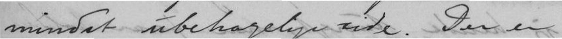mindst ubehagelige side. Der er
